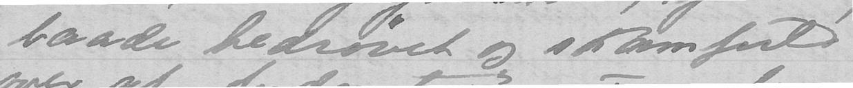baade bedrøvet og skamfuld
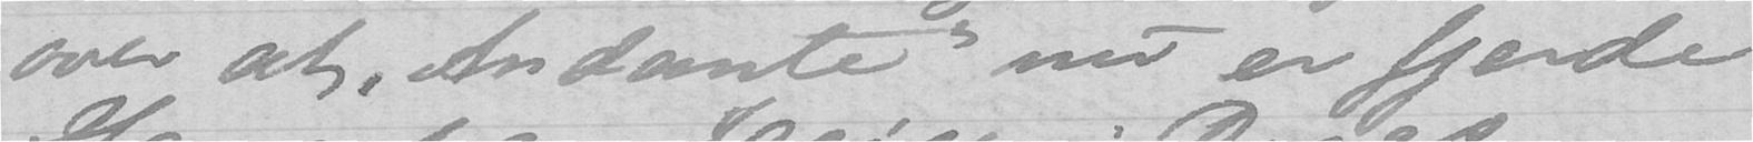over at "Andante" nu er fjerde
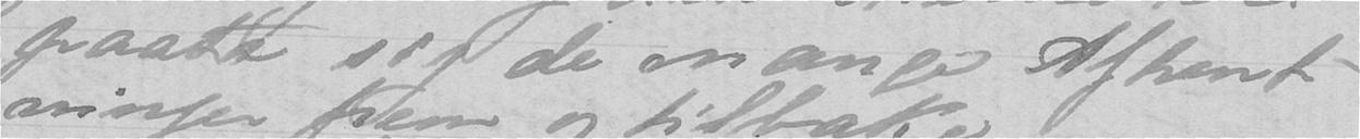paata sig de mange Afhent-
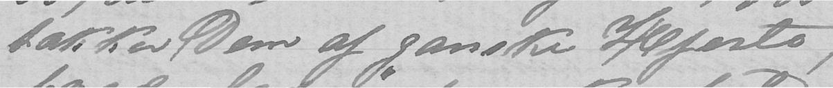takker Dem af ganske Hjerte,
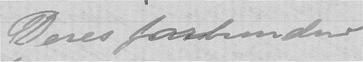Deres forbundne
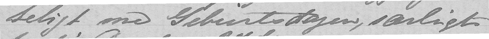teligt med Geburtsdagen, særligt
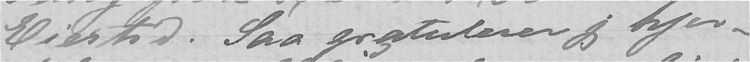Eiertid. Saa gratulerer jeg hjer-
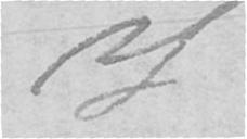H
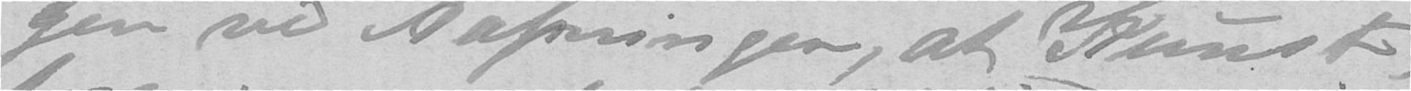gen ved Aapningen, at Kunst-
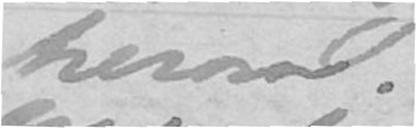herom.
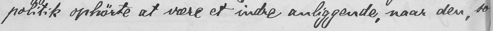politik ophørte at være et indre anliggende, naar den, som
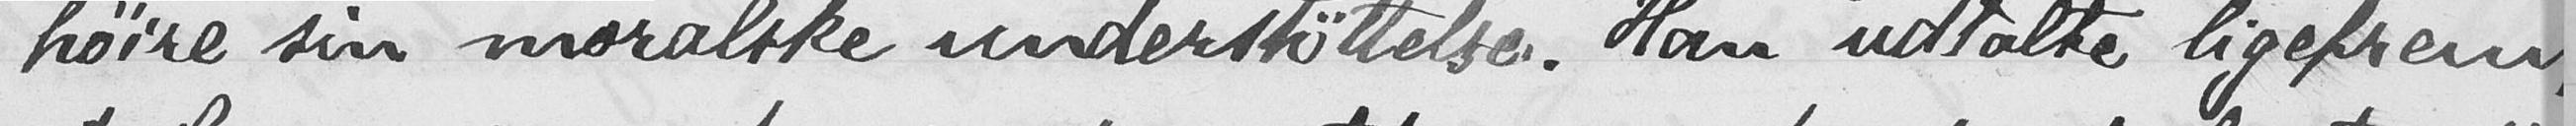høire sin moralske understøttelse. Han udtalte ligefrem,
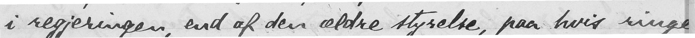i regjeringen, end af den ældre styrelse, paa hvis ringe
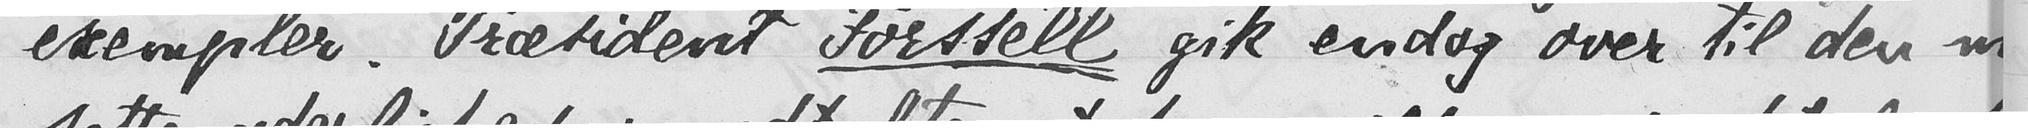exempler. Præsident Forssell gik endog over til den mod-
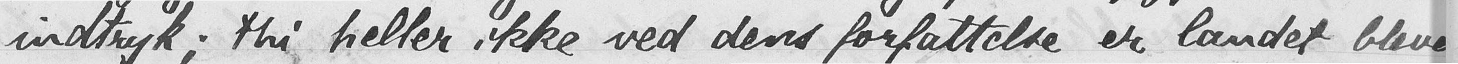indtryk; thi heller ikke ved dens forfattelse er landet blevet
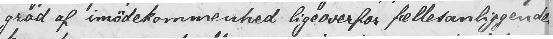grad af imødekommenhed ligeoverfor fællesanliggenderne,
